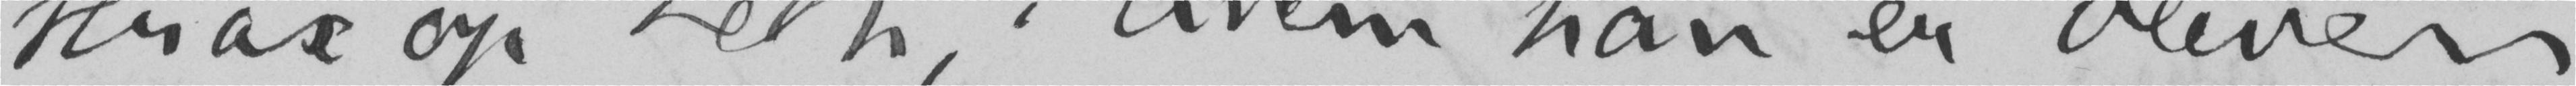strax op Leth, i hvem han er bleven
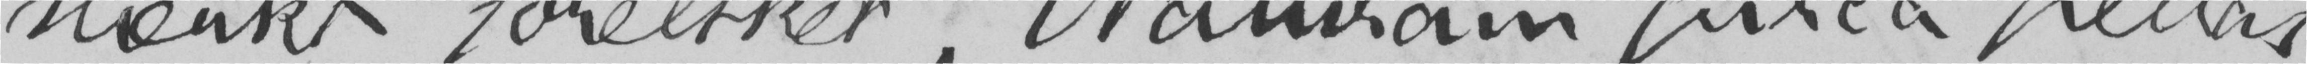stærkt forelsket. Naturam furca pellas
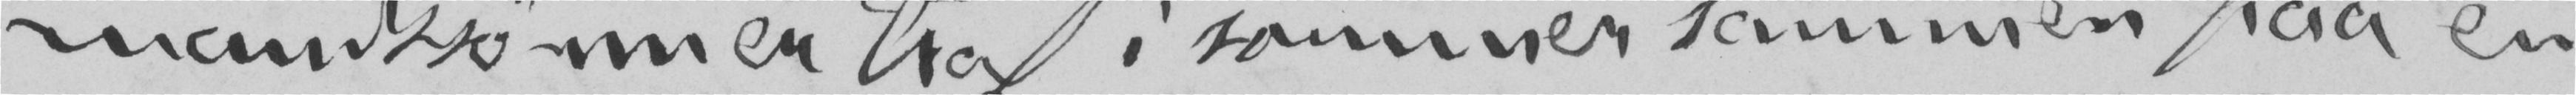mandssønner traf i sommer sammen paa en
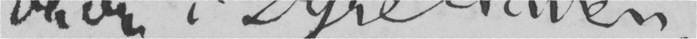bror i Dyrehaven.
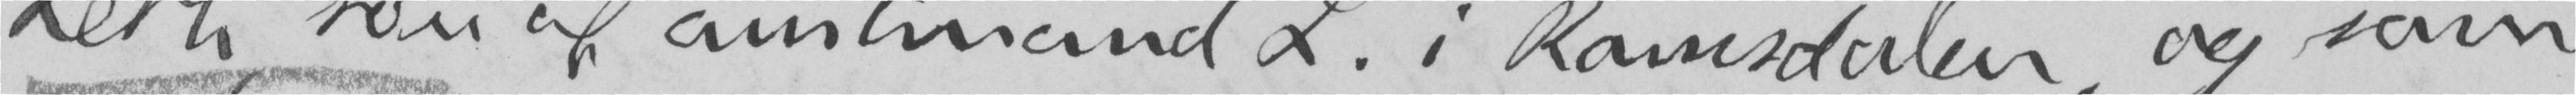Leth, søn af amtmand L. i Romsdalen, og som
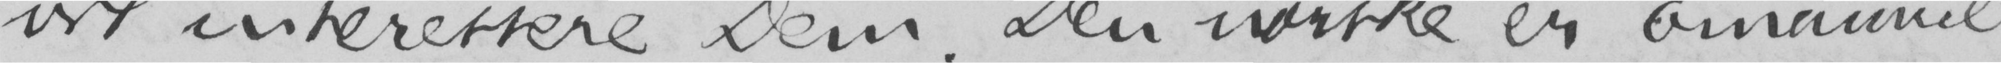vil interessere Dem. Den norske er Emanuel
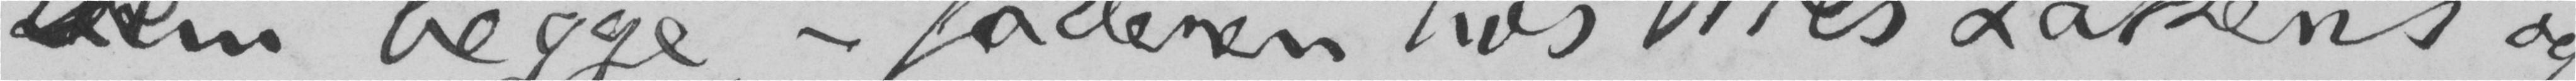dem begge, – faderen hos Nils Lassen’s og
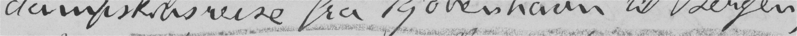dampskibsreise fra Kjøbenhavn til Bergen,
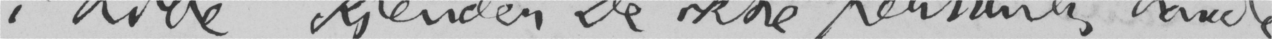i Ribe. Kjender De ikke personlig <…??>
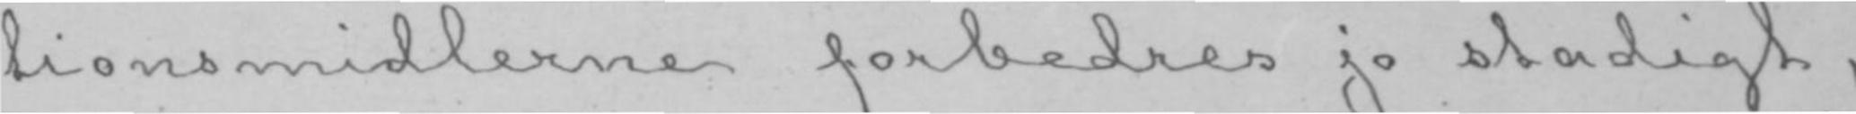tionsmidlerne forbedres jo stadigt,
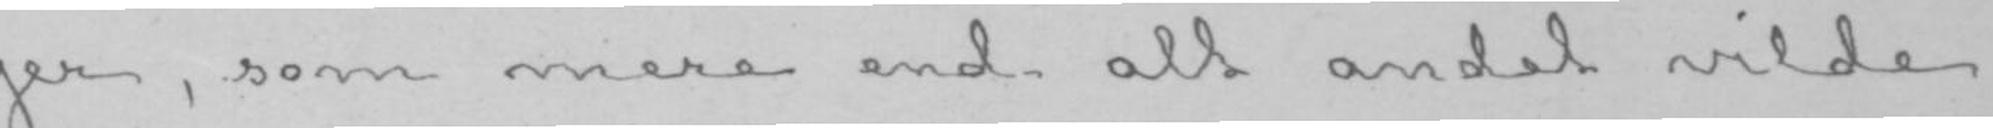ger, som mere end alt andet vilde
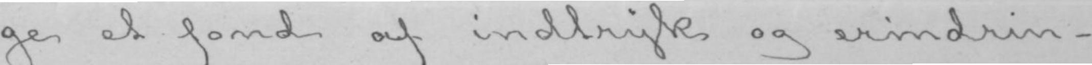ge et fond af indtryk og erindrin-
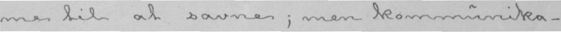me til at savne; men kommunika-
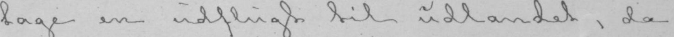tage en udflugt til udlandet, da
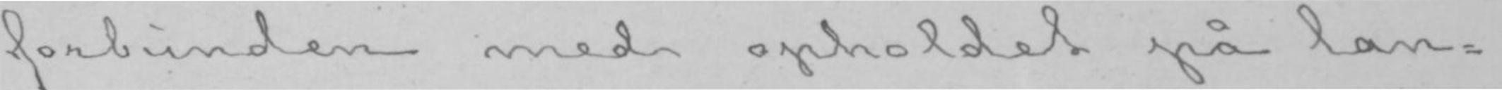forbunden med opholdet på lan-
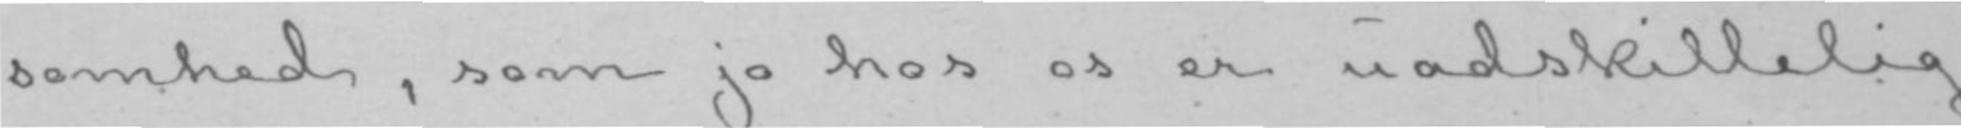somhed, som jo hos os er uadskillelig
